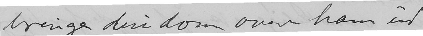bringe din dom over ham ud
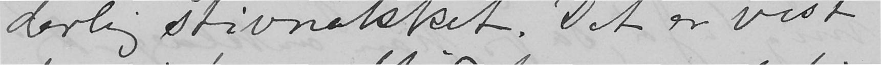derlig stivnakket. Det er vist
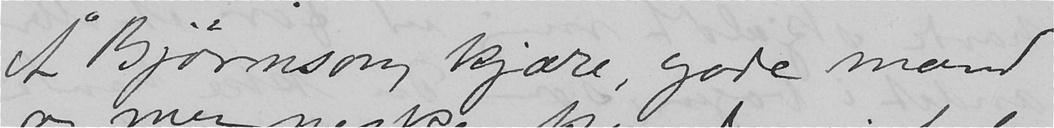Å Bjørnson, kjære, gode mand
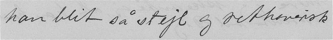han blit så stejl og rethaverisk
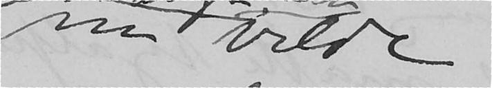nu vilde
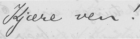Kjære ven!
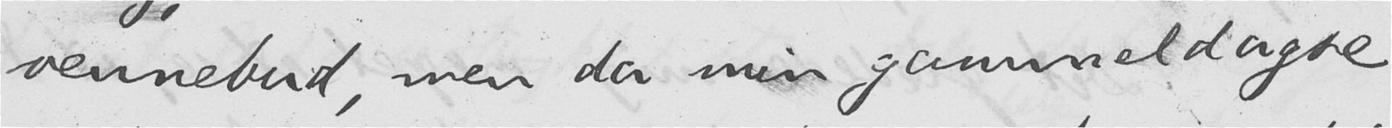vennebud, men da min gammeldagse
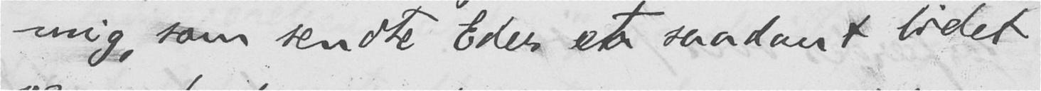mig, som sendte Eder et saadant lidet
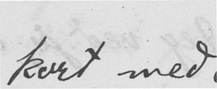kort med
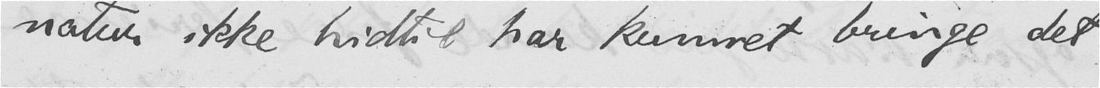natur ikke hidtil har kunnet bringe det
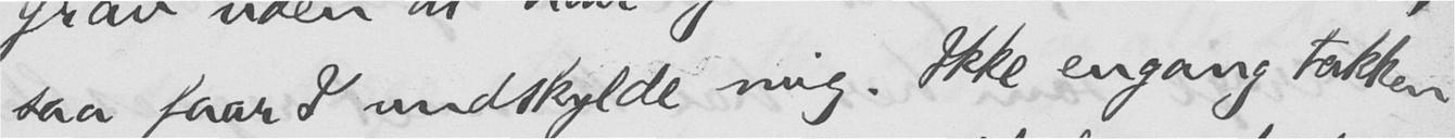saa faar I undskylde mig. Ikke engang takken
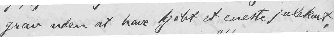grav uden at have kjøbt et eneste julekort,
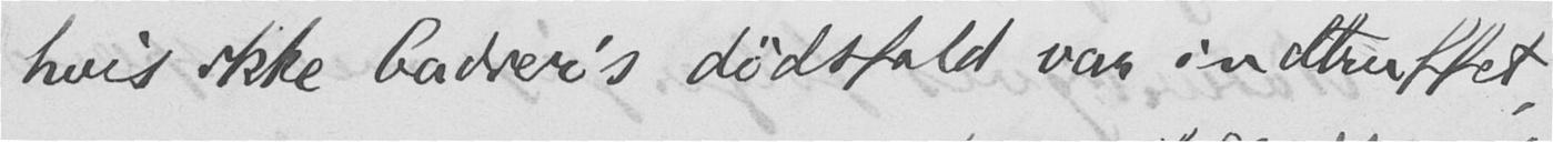hvis ikke Gadser’s dødsfald var indtruffet,
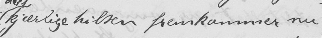kjærlige hilsen fremkommer nu
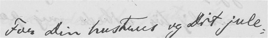For Din hustrus og dit jule-
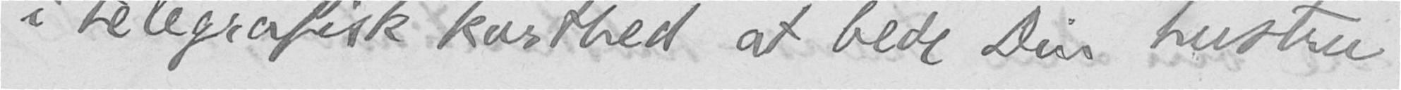i telegrafisk korthed at bede Din hustru -
